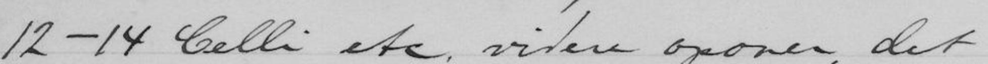12-14 Celli etc. videre opover, det
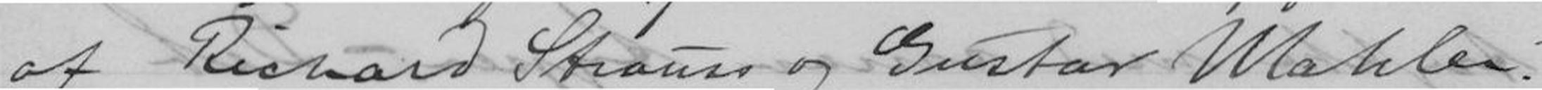af Richard Strauss og Gustav Mahler.
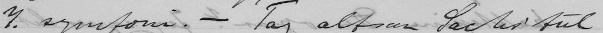7. symfoni. - Tag altsaa Sachs tul
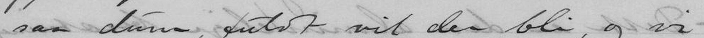saa dum, fuldt vil der bli, og vi
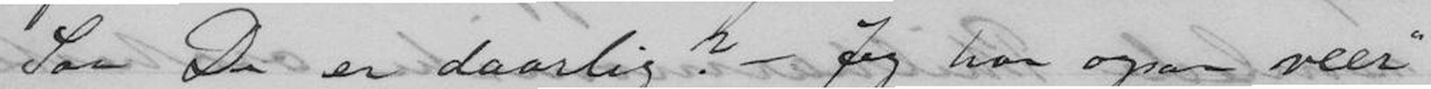Saa De er daarlig? - Jeg har ogsaa "veer"
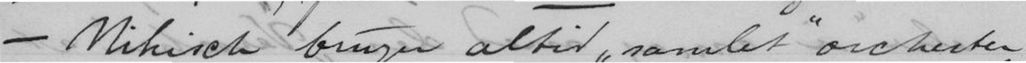- Nikisch bruger altid "samlet" orchester,
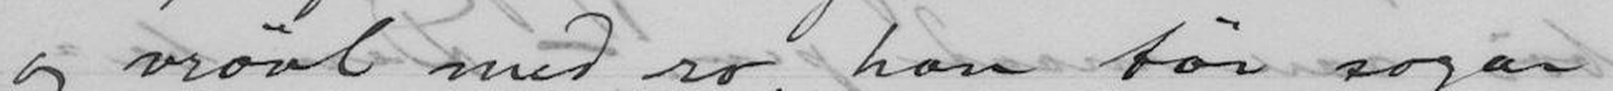og vrøvl med ro, han tør sogar
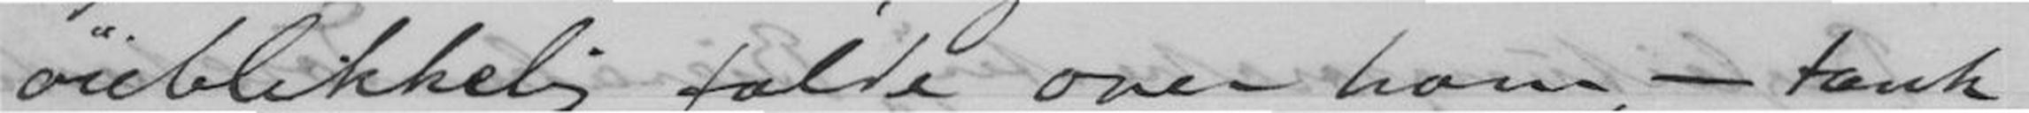øieblikkelig falde over ham, - tænk
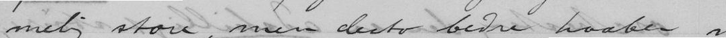melig store, men desto bedre haaber
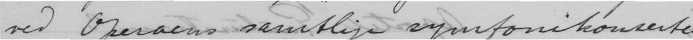ved Operaens samtlige symfonikonserter
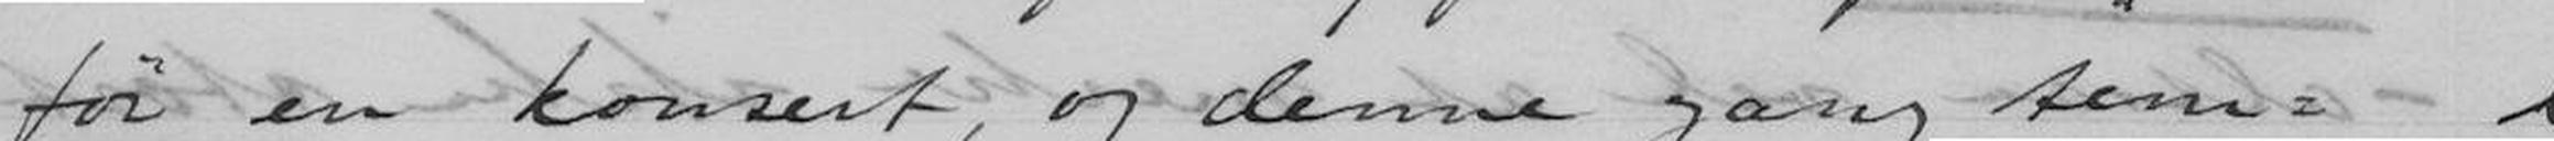før en konsert, og denne gang tem-
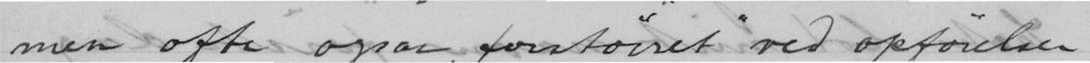men ofte ogsaa "forstørret" ved opførelsen
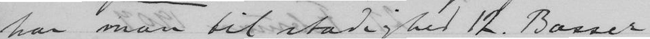har man til stadighed 12. Basser
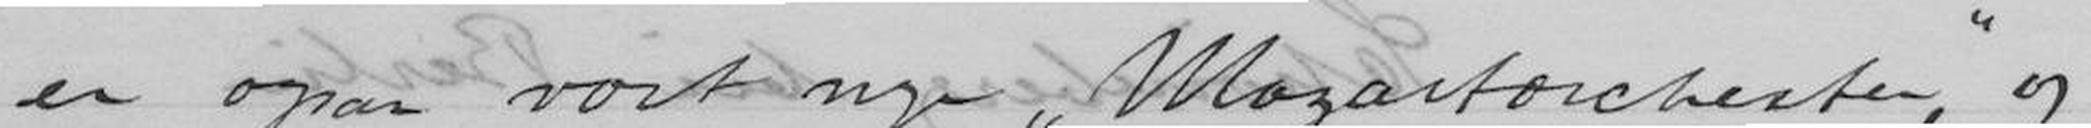er ogsaa vort nye "Mozartorchester", og
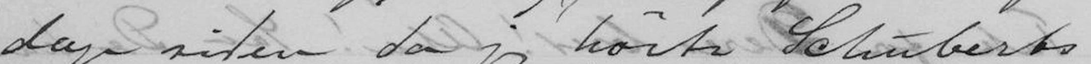dage siden da jeg hørte Schuberts
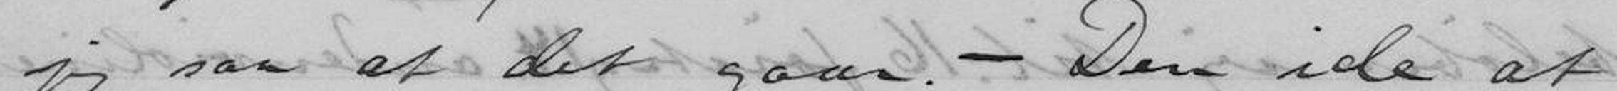jeg saa at det gaar. - Den ide at
